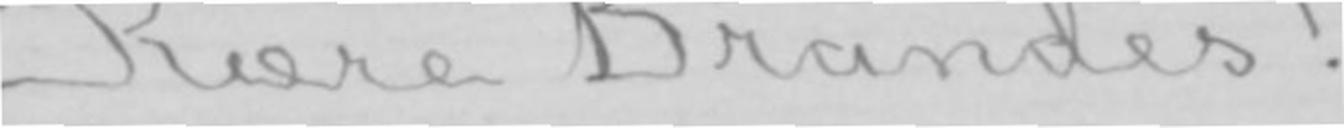Kære Brandes!
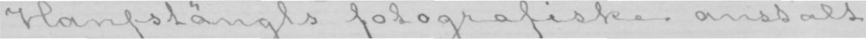Hanfstängls fotografiske anstalt
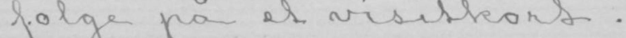følge på et visitkort.
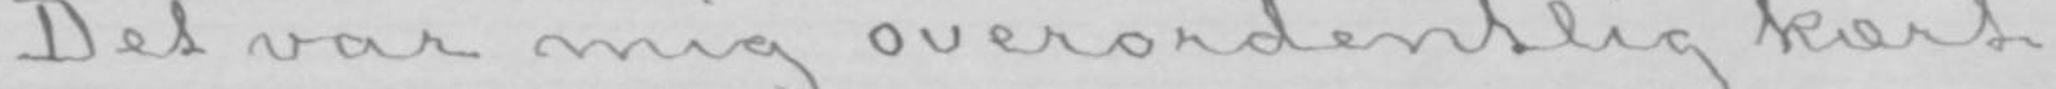Det var mig overordentlig kært
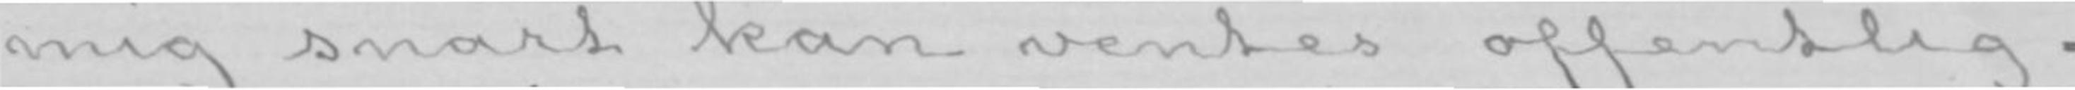mig snart kan ventes offentlig-
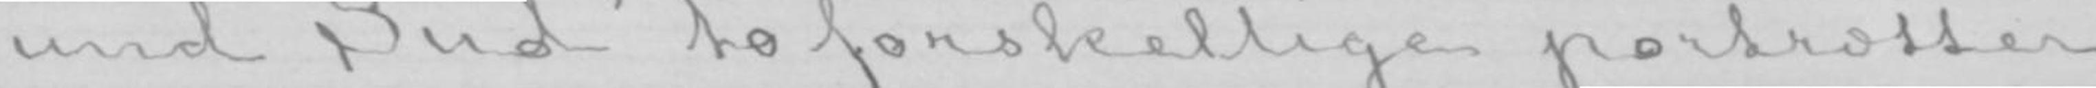und Süd» to forskellige portrætter
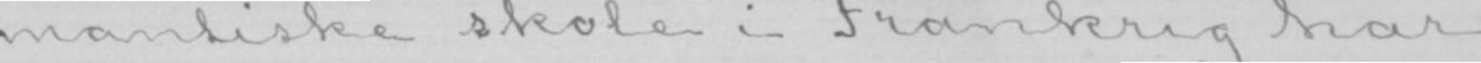mantiske skole i Frankrig har
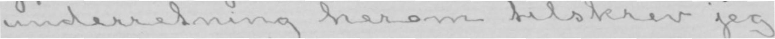underretning herom tilskrev jeg
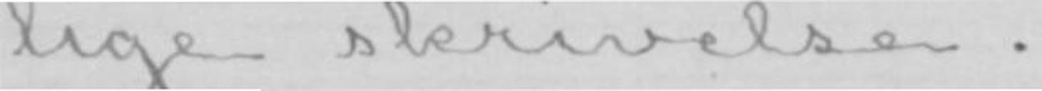lige skrivelse.
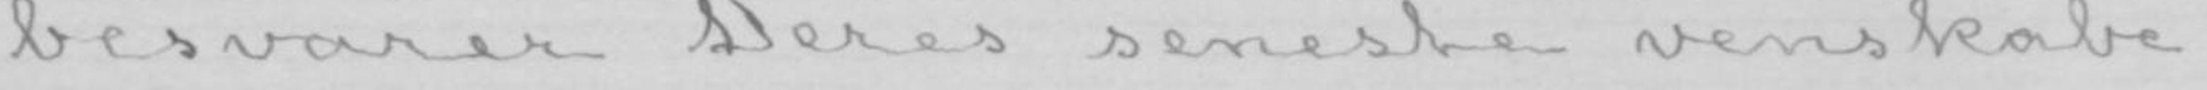besvarer Deres seneste venskabe-
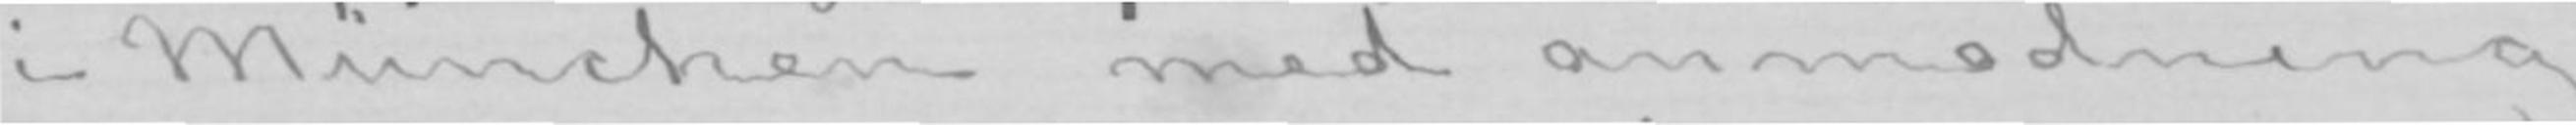i München med anmodning
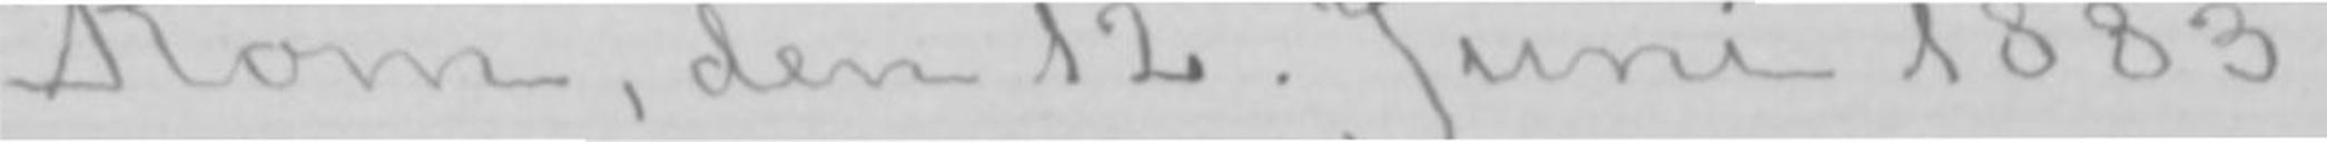Rom, den 12. Juni 1883.
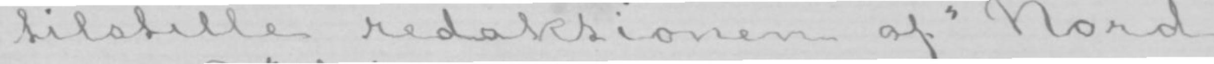tilstille redaktionen af «Nord
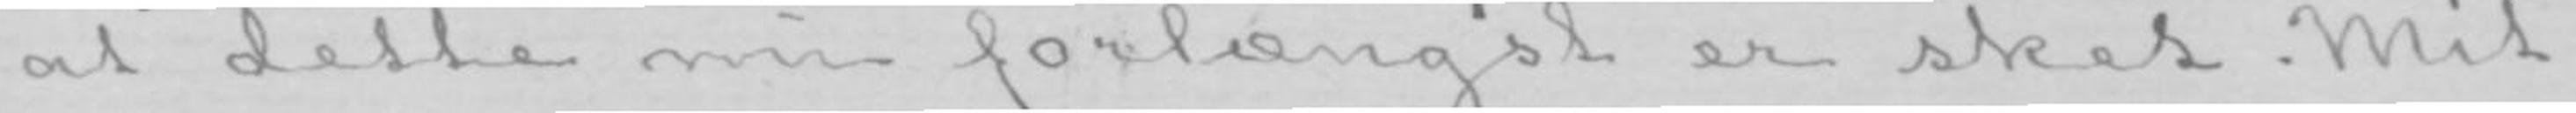at dette nu forlængst er sket. Mit
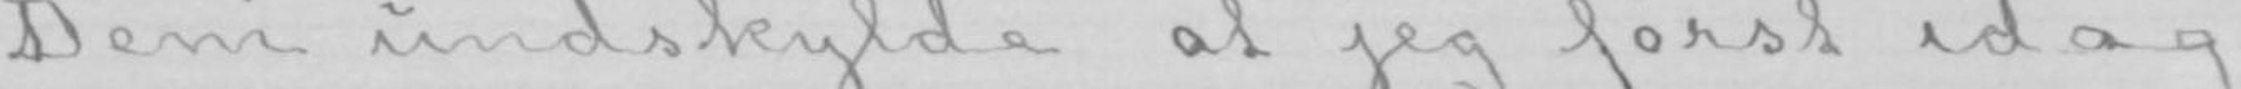Dem undskylde at jeg først idag
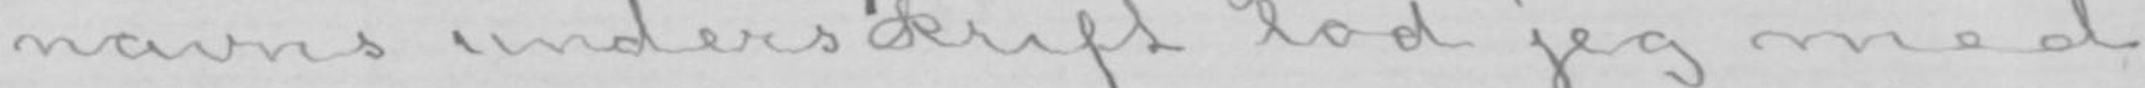navns underskrift lod jeg med-
